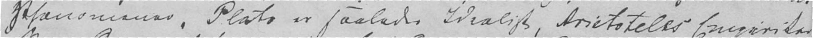Phænomener. Plato er saaledes Idealist, Aristoteles Empiriker.
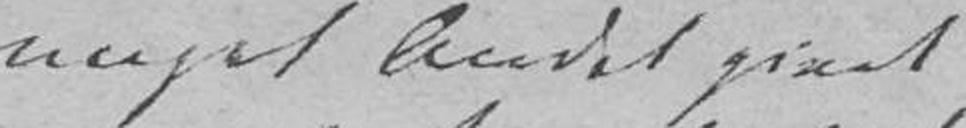meget Andet givet
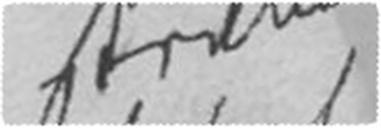stræbe
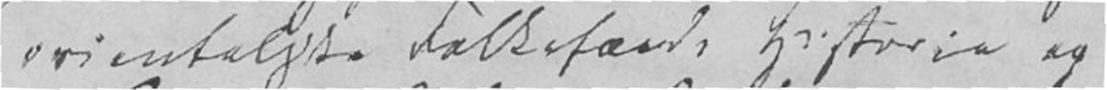orientalske Folkefærds Historie og
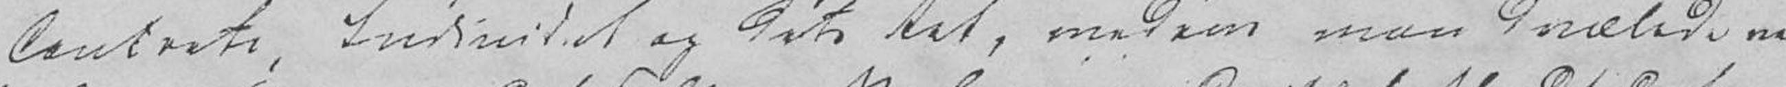Concrete, Individet og dets Ret, medens man dvælede ved
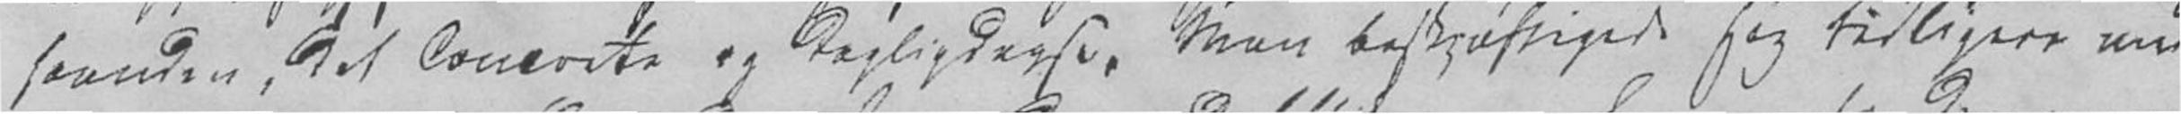haanden: det Concrete og Dagligdagse. Man beskjæftigede sig tidligere med
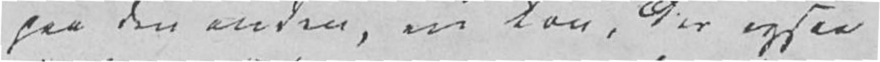paa den anden, en Lov, der ogsaa
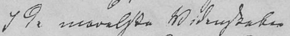I de moralske Videnskaber
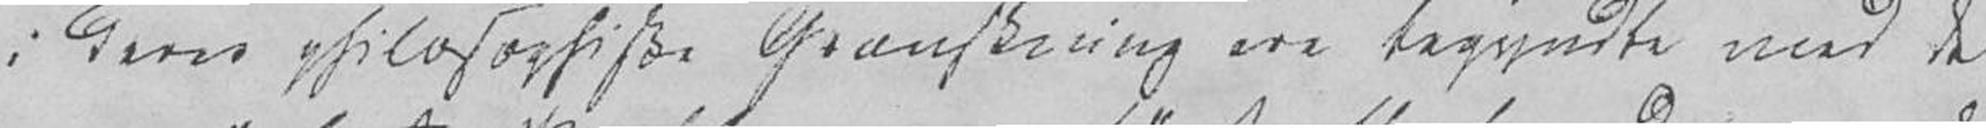i deres philosophiske Granskning ere begyndte med de
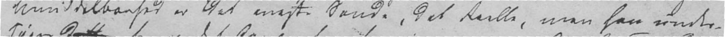Umiddelbarhed er det eneste Sande, det Reelle, men han under-
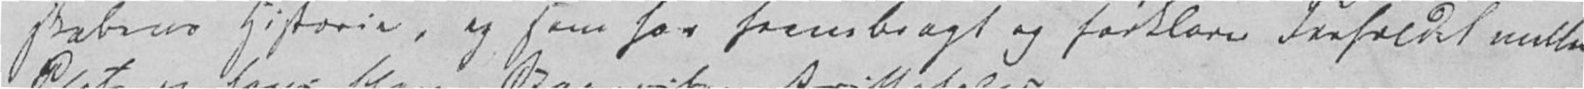skabens Historie, og som har frembragt og forklarer Forholdet mellem
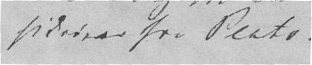hidhører fra Plato.
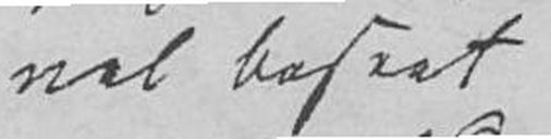vel beseet
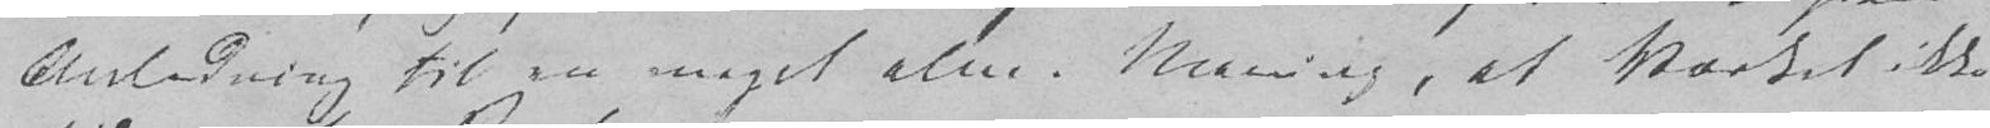Anledning til en meget alm. Mening, at Værket ikke
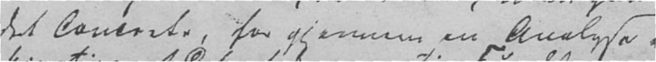det Concrete, for gjennem en Analyse
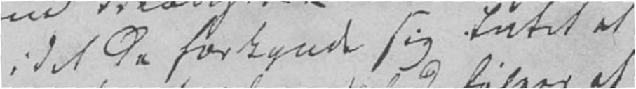idet de forkynde sig Intet at
